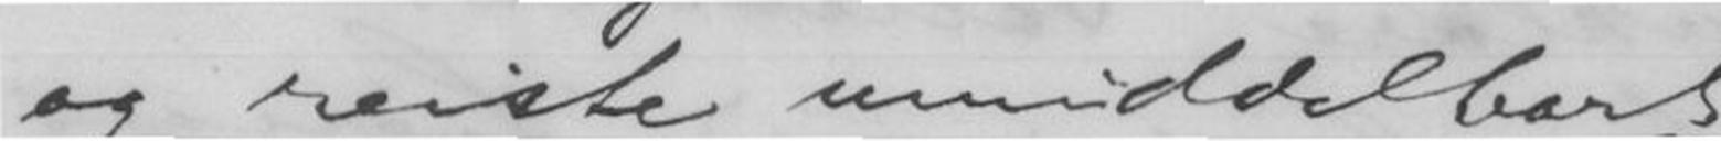og reiste umiddelbart
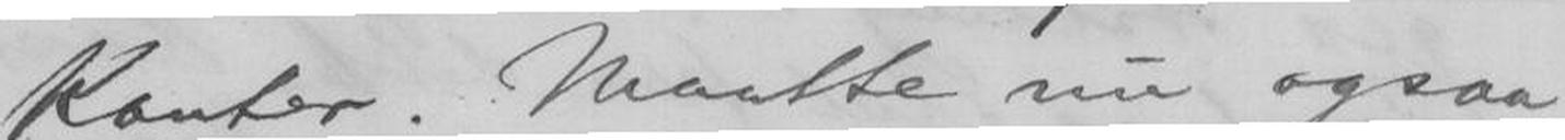Kanter. Maatte nu ogsaa
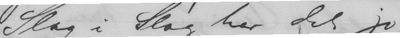Slag i Slag har det jo
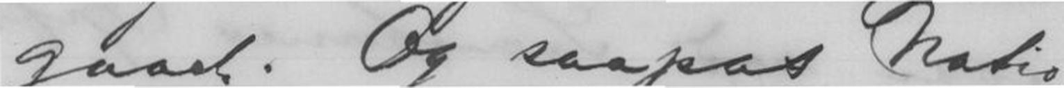gaaet. Og saapas Natio-
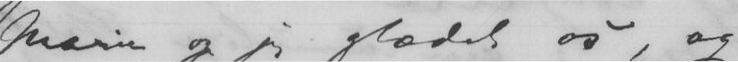Marie og jeg glædet os, og
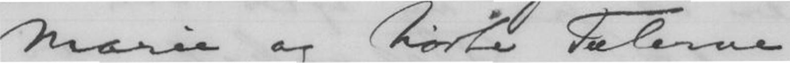Marie og hørte Talerne,
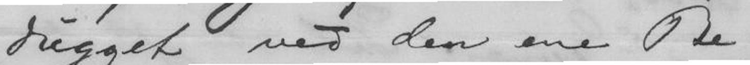dugget ved den ene Be-
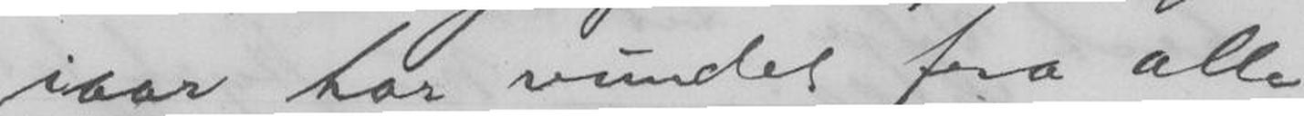iaar har vundet fra alle
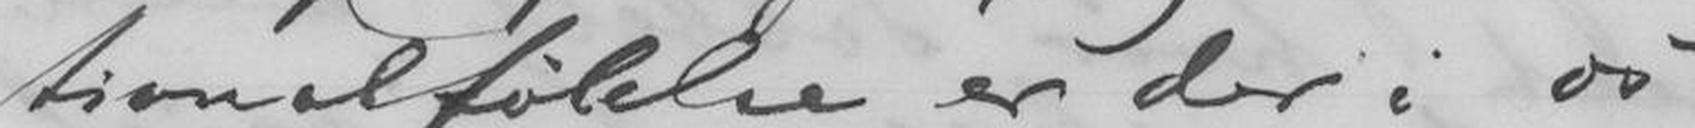tionalfølelse er der i os,
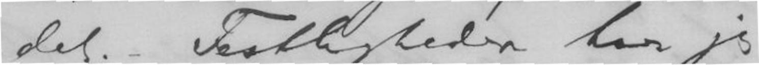det. Festligheden har jeg
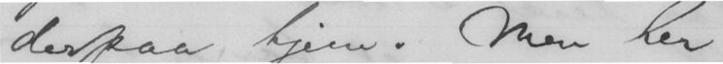derpaa hjem. Men her-
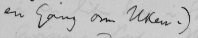en Gang om Uken.)
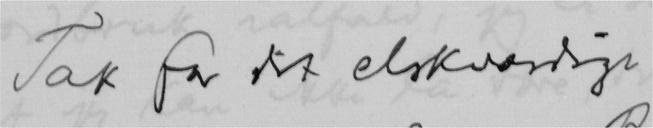Tak for det elskværdige
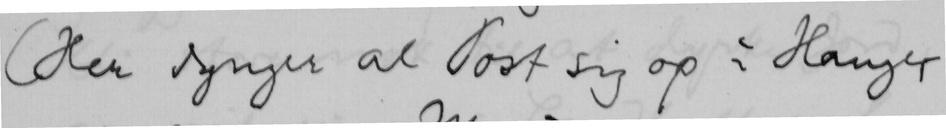(Her dynger al Post sig op i Hauger
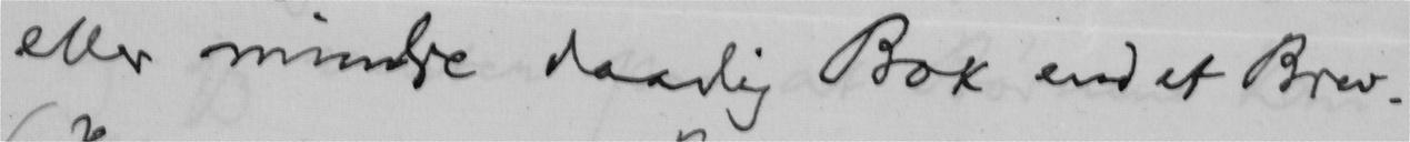eller mindre daarlig Bok end et Brev.
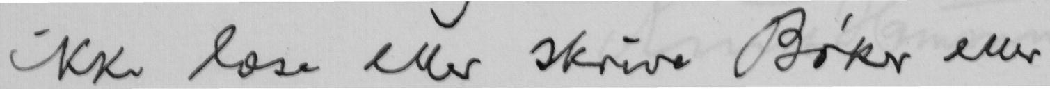ikke læse eller skrive Bøker eller
